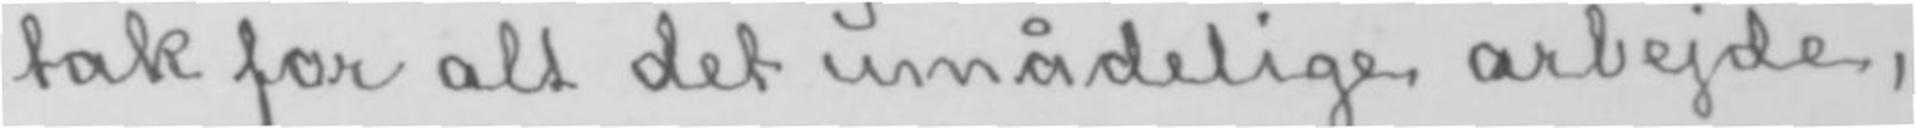tak for alt det umådelige arbejde,
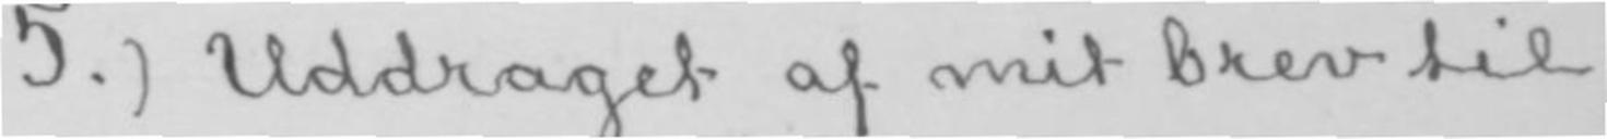5.) Uddraget af mit brev til
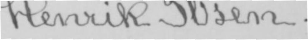Henrik Ibsen.
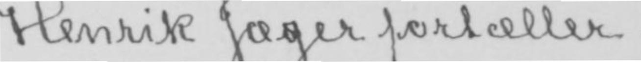Henrik Jæger fortæller.
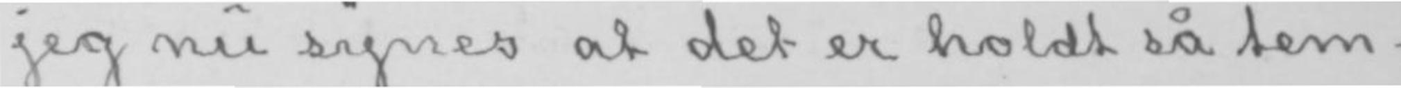jeg nu synes at det er holdt så tem-
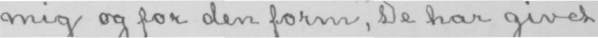mig og for den form, De har givet
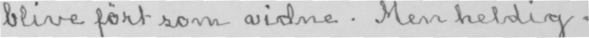blive ført som vidne. Men heldig-
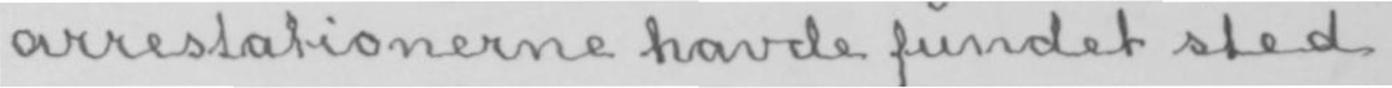arrestationerne havde fundet sted
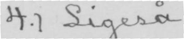4.) Ligeså.
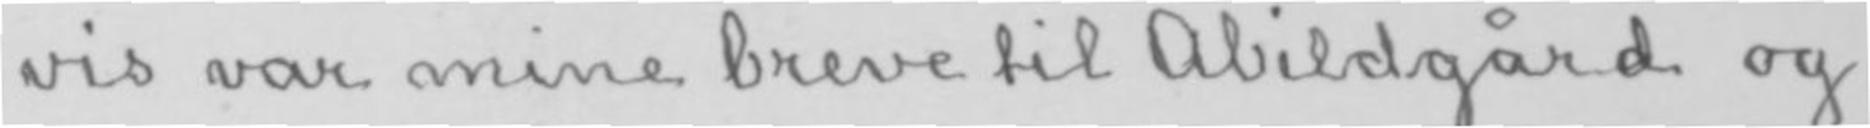vis var mine breve til Abildgård og
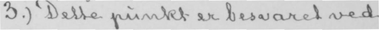3.) Dette punkt er besvaret ved
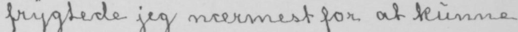frygtede jeg nærmest for at kunne
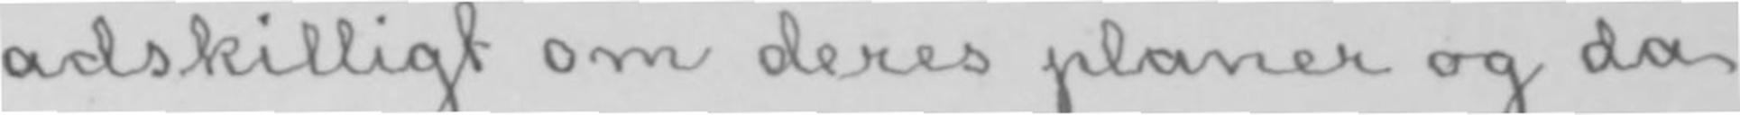adskilligt om deres planer og da
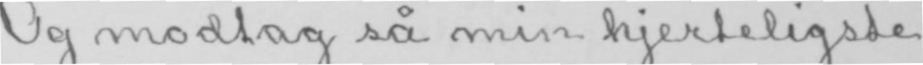Og modtag så min hjerteligste
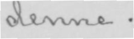denne.
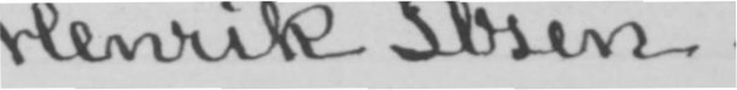Henrik Ibsen.
